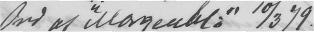Ord af "Morgenbls" 10/3 79.
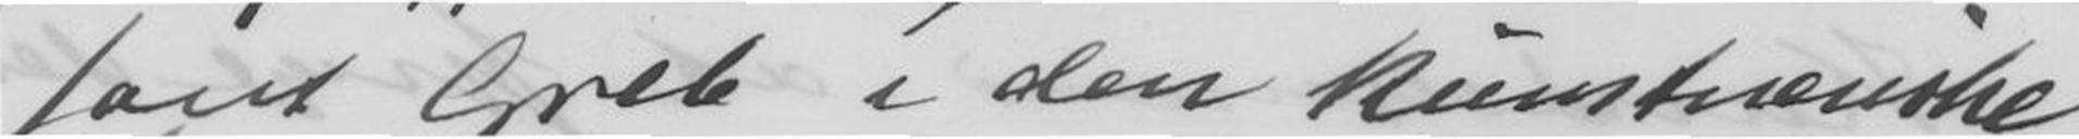sant Greb i den kunstneriske
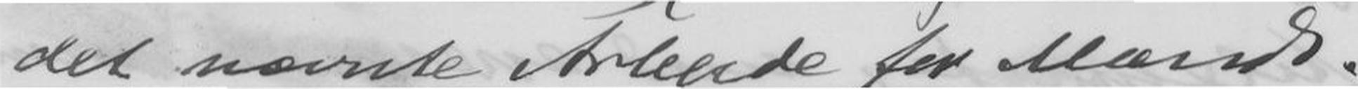det nævnte Arbeide for Mand-
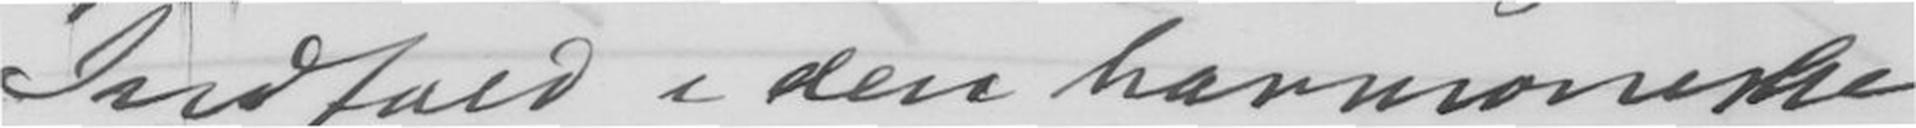Indfald i den harmoniske
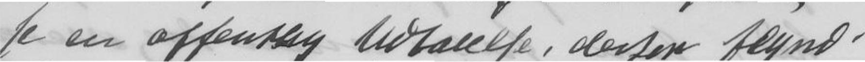se en offentlig Udtalelse, derfor flgnd'
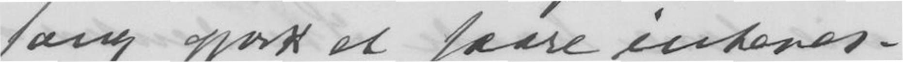sang gjort et saare interes-
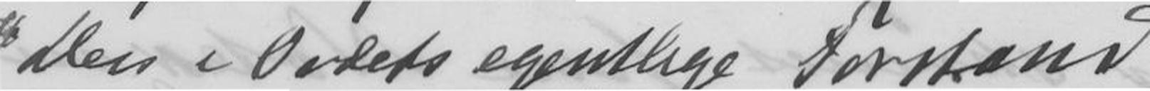"Den i Ordets egentlige Forstand
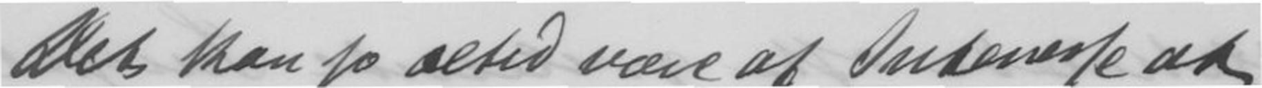Det kan jo altid være af Interesse at
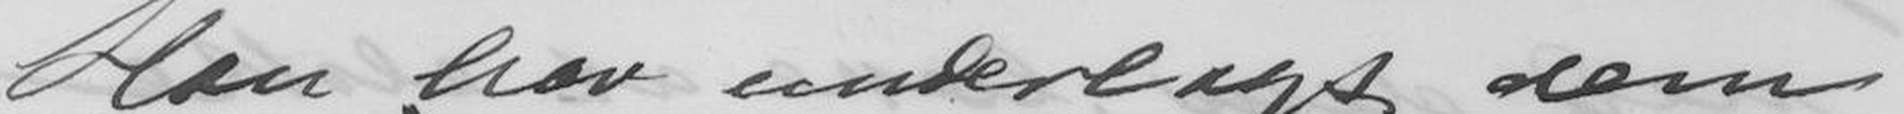Han har underlagt dem
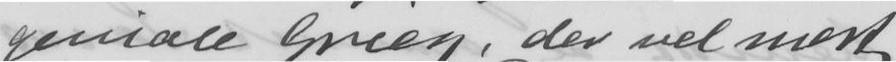geniale Grieg, der vel mest
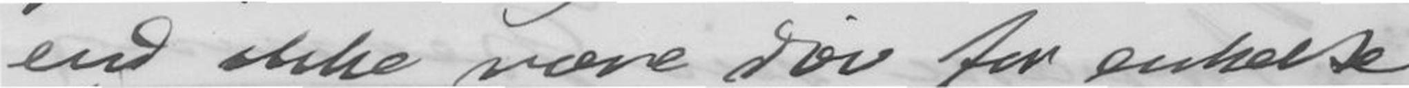end ikke være Døv for enkelte
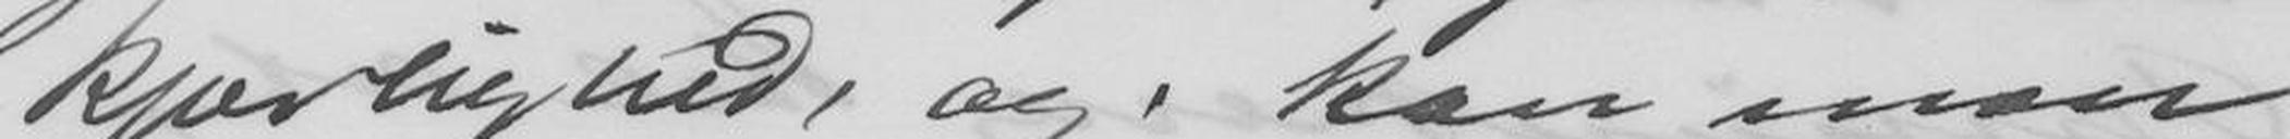kjærlighed, og kan man
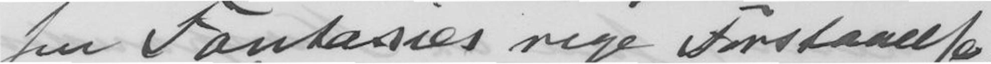sin Fantasies rige Forstaaelse
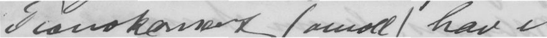Pianokonsert (amoll) har i
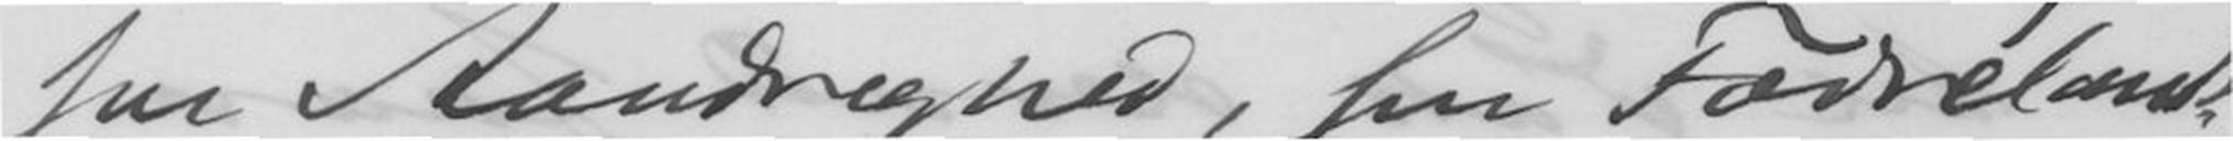for Aandraghed, sin Fædrelands-
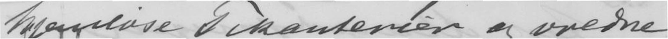hjemløse Pekanterier og vrede
